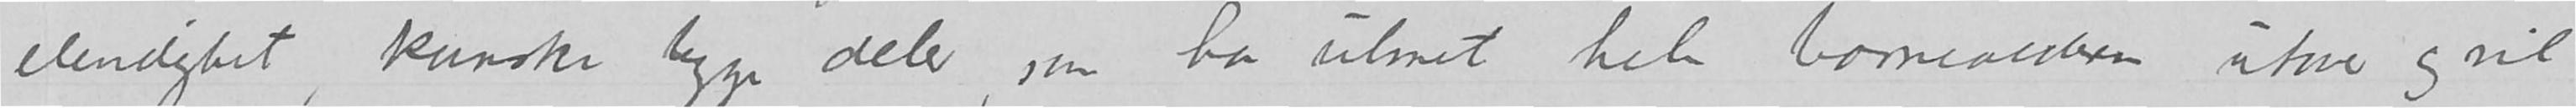elendighet, kanske begge deler, som har ulmet hele barnealderen utover og vil
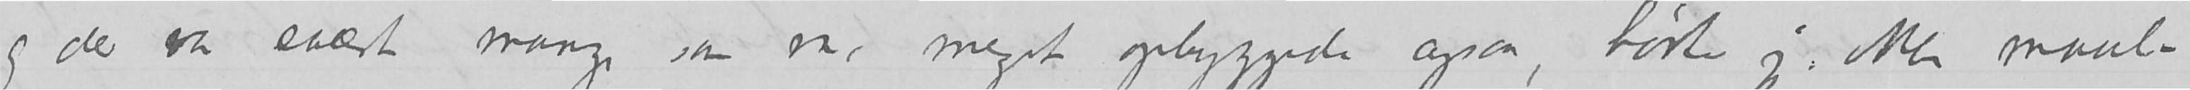og der var svært mange som var meget opbyggede ogsaa, hørte jeg.  Men maalfolket
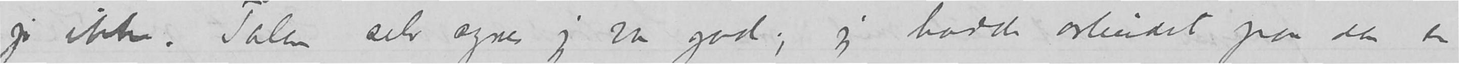jo ikke.  Talen selv synes jeg var god; jeg hadde arbeidet paa den en
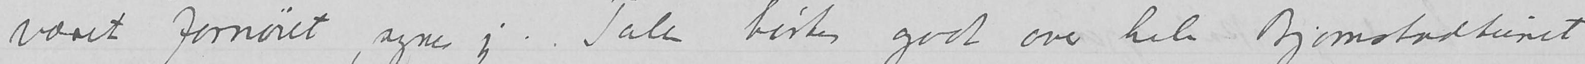været fornøiet, synes jeg.  Talen hørtes godt over hele Bjørnstadtunet,
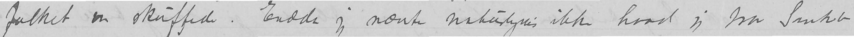var skuffede.  Endda jeg nævnte naturligvis ikke hvad jeg tror Sankt
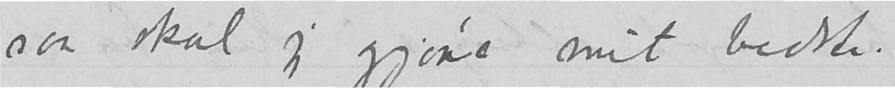saa skal jeg gjøre mit bedste.
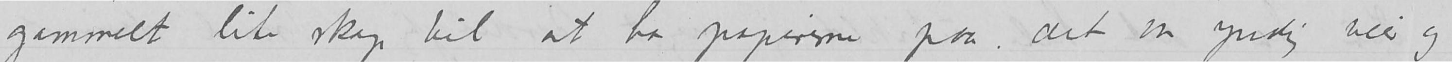gammelt lite skap til at ha papirerne paa;  det var yndig veir og
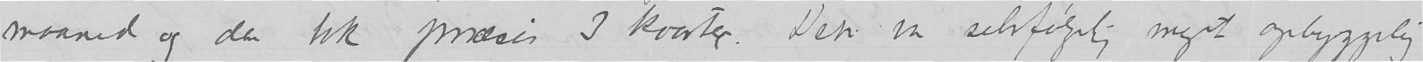maaned og den tok præsis 3 kvarter.  Den var selvfølgelig meget opbyggelig
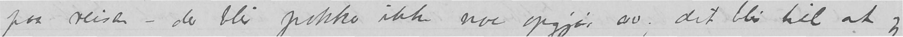paa reisen – der blir pokker ikke noe opgjør av; det blir til at jeg
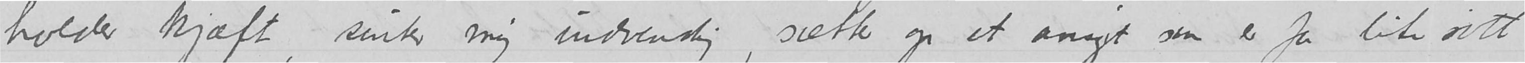holder kjæft, sinter mig indvendig, sætter op et ansigt som er for lite søtt
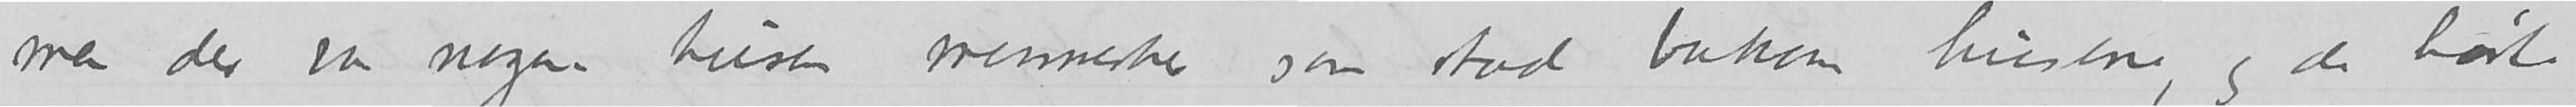men der var nogen tusen mennesker som stod bakom husene, og de hørte
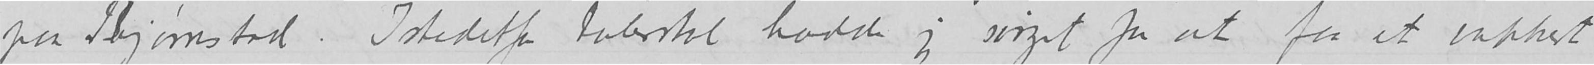paa Bjørnstad.  Istedetfor talerstol hadde jeg sørget for at faa et vakkert
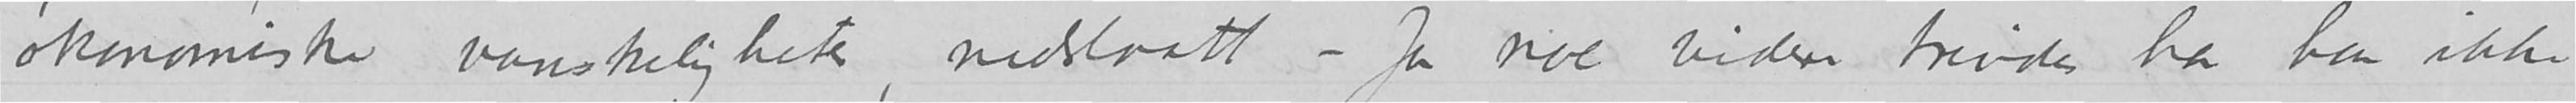økonomiske vanskeligheter, nedslaatt – ja noe videre trivdes har han ikke
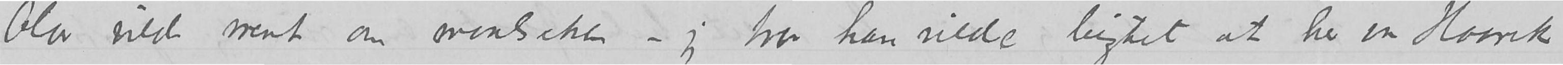Olav vilde ment om maalsaken – jeg tror han vilde lugtet at her var Haarek
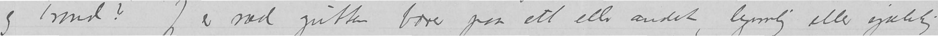og Trond?  Jeg er ræd gutten bærer paa ett eller andet, legemlig eller sjælelig
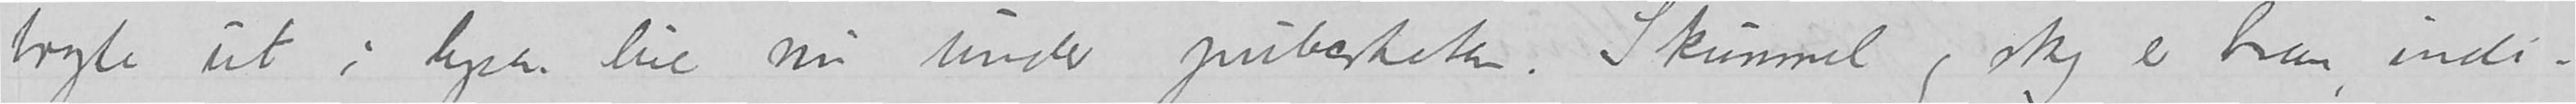bryte ut i lyse live nu under puberteten.  Skummel og sky er han, indi-
